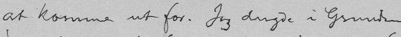at komme ut for. Jeg dugde i Grunden
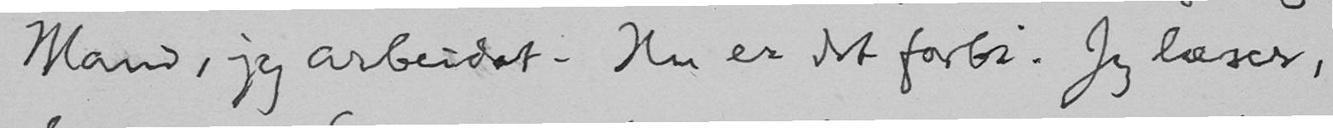Mand, jeg arbeidet. Nu er det forbi. Jeg læser,
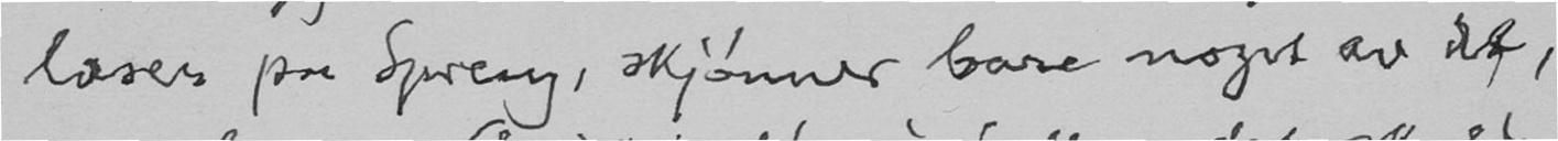læser paa Spreng, skjønner bare noget av det,
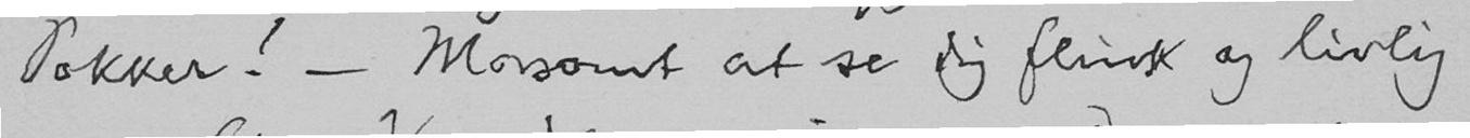Pokker! - Morsomt at se dig flink og livlig
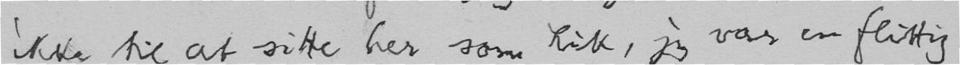ikke til at sitte her som Lik, jeg var en flittig
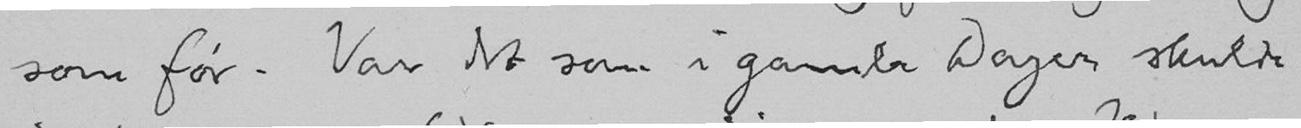som før. Var det som i gamle Dager skulde
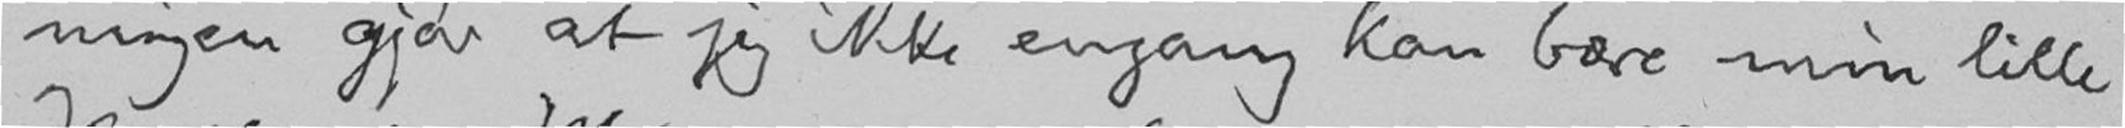ningen gjør at jeg ikke engang kan bære min lille
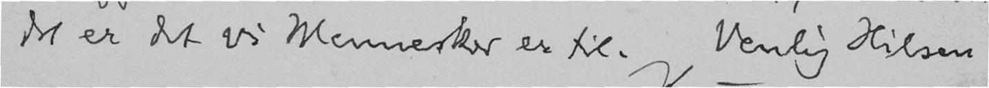det er det vi Mennesker er til. Venlig Hilsen
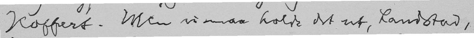Koffert. Men vi maa holde det ut, Landstad,
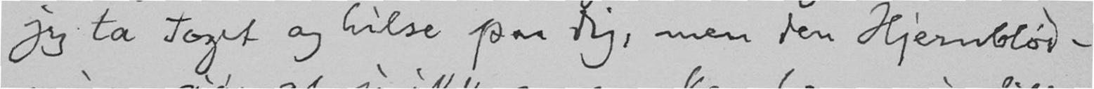jeg ta Toget og hilse paa dig, men den Hjernblød-
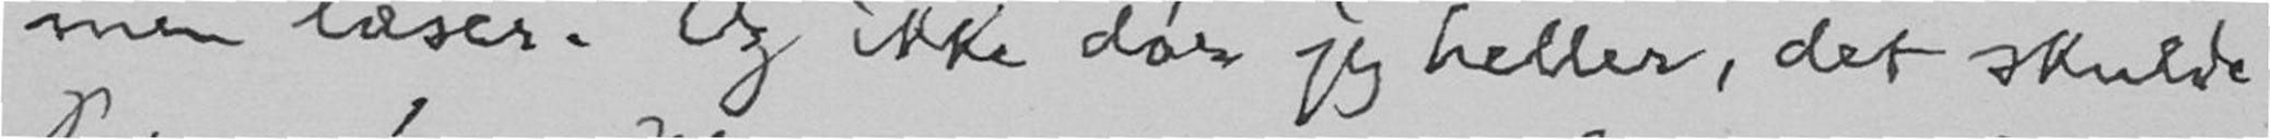men læser. Og ikke dør jeg heller, det skulde
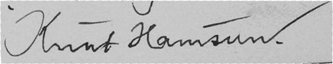Knut Hamsun.
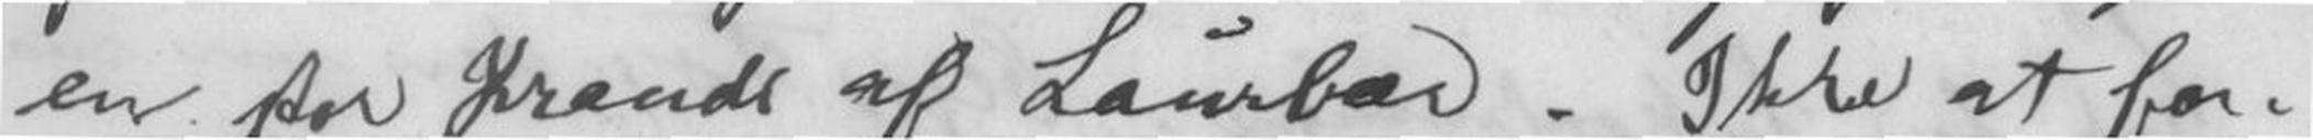en stor Krands af Laurbær. Ikke at for-
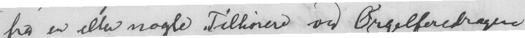fra en eller nogle Tilhørere ved Orgelforedrager
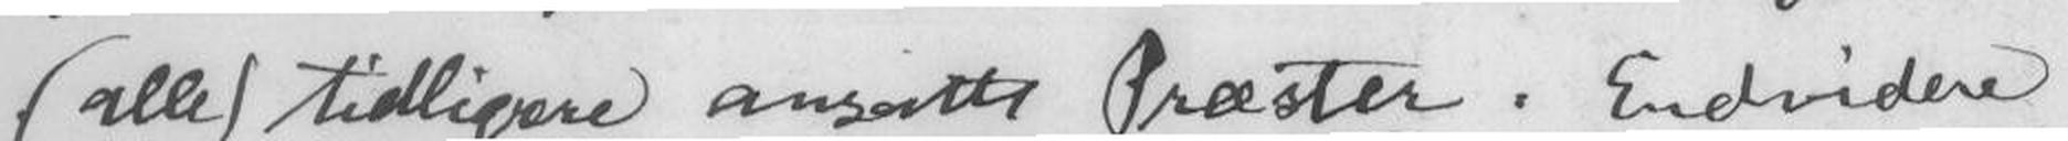(alle) tidligere ansatte Præster. Endvidere
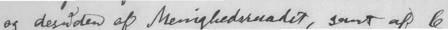og desuden af Menighedsraadet, samt af 6
